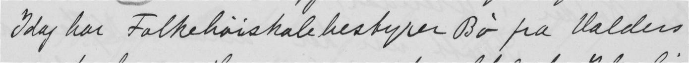Idag har Folkehøiskolebestyrer Bø fra Valders
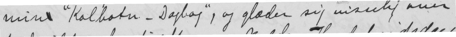min "Kolbotn - Dagbog", og glæder sig visselig over
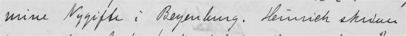mine Nygifte i Beyenburg. Heinrich skriver
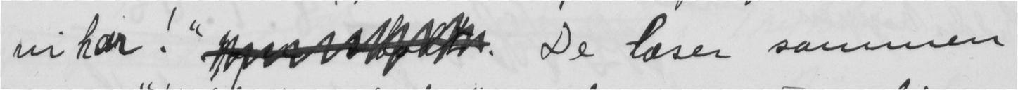vi har!"  De læser sammen
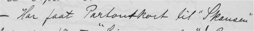– Har faat Partontkort til "Skansen"
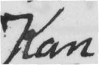Kan
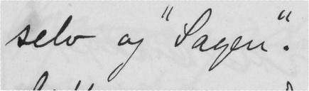selv og "Sagen".
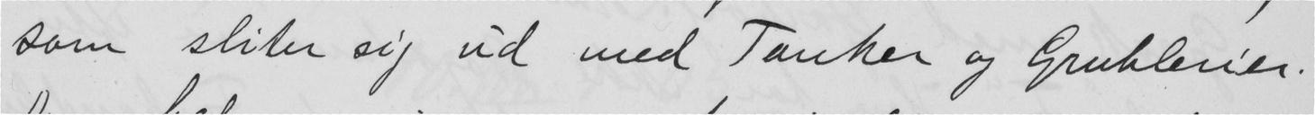som sliter sig ud med Tanker og Grublerier.
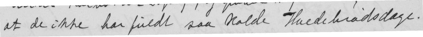at de ikke har fuldt saa kolde Hvedebrødsdage.
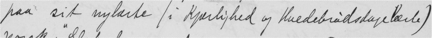paa sit nylærte (i Kjærlighed og Hvedebrødsdagelærte)
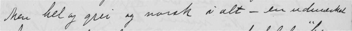Men hel og grei og norsk i alt - en udmærket
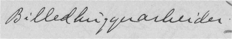Billedhuggerarbeider.
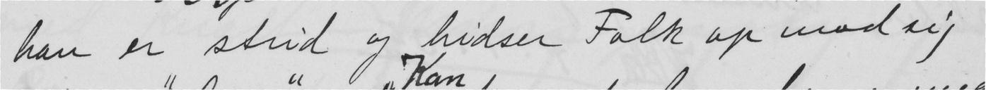han er strid og hidser Folk op mod sig
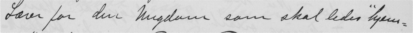Lærer for den Ungdom som skal ledes "hjem-
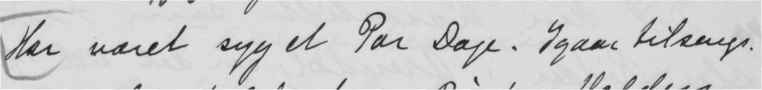Har været syg et Par Dage. Igaar tilsengs.
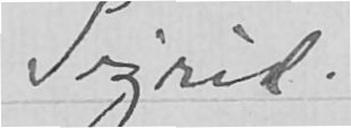Sigrid.
